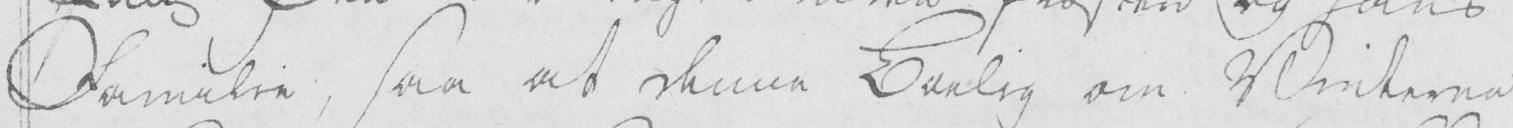Familie, saa at denne Boelig om Vinteren
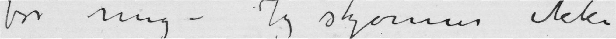for mig – Jeg skjønner ikke
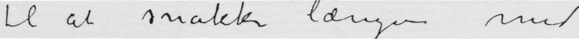til at snakke længer med
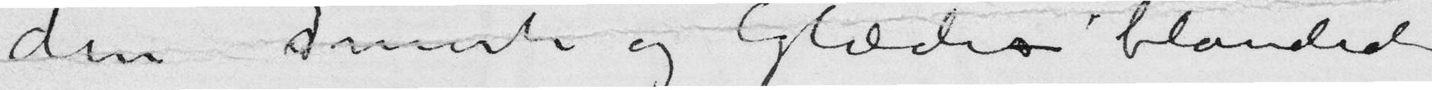den Smerte og Glædesblandede
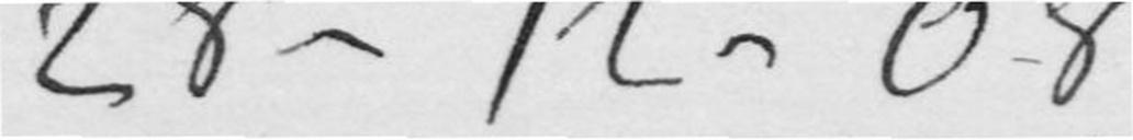28-12-08
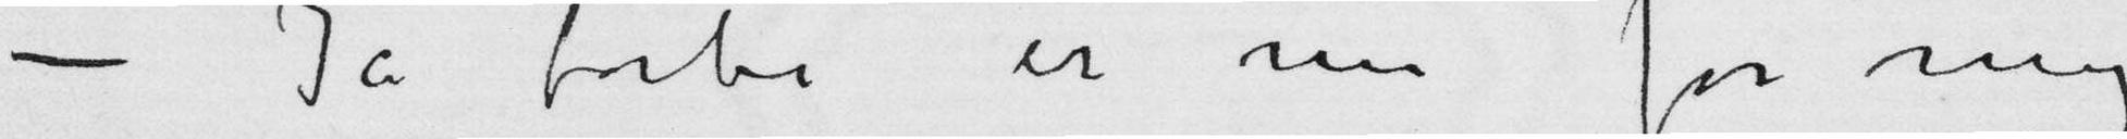– Ja forbi er nu for mig
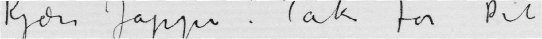Kjære Jappe! Tak for Dit
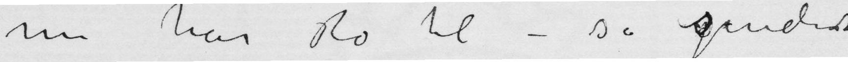nu har Ro til – så sender
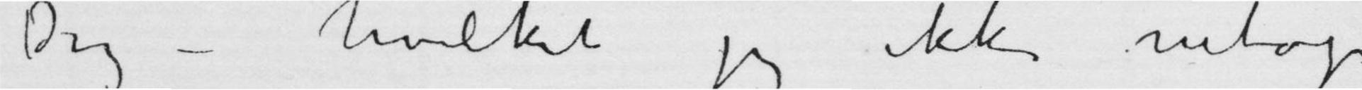Dig – hvilket jeg ikke netop
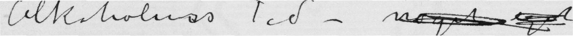Alkoholens Tid – noget eget
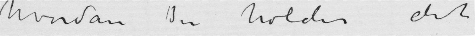hvordan du holder det
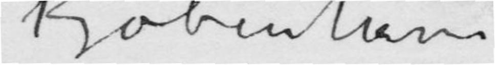Kjøbenhavn
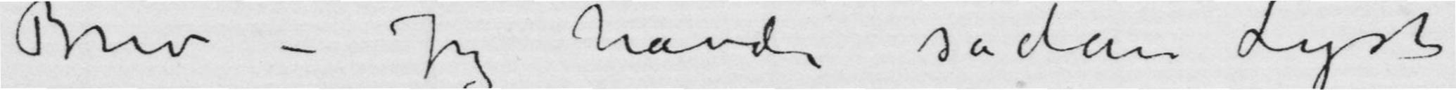Brev – Jeg havde sådan Lyst
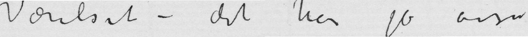Værelset – det har jo osså
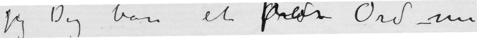jeg dig bare et par Ord –nu
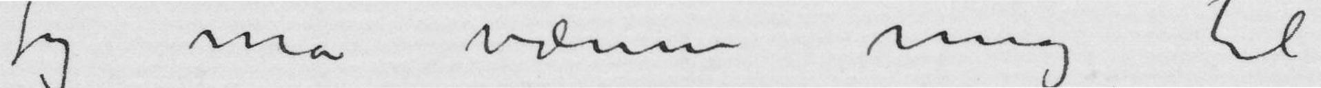Jeg må vænne mig til
